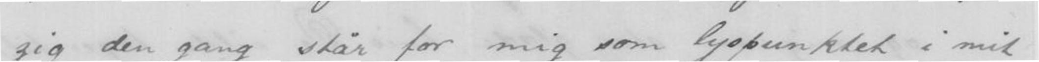zig den gang står for mig som lyspunktet i mit
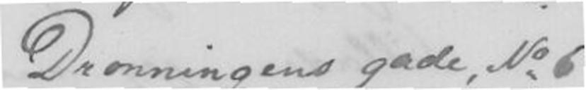Dronningensgade, N. 6
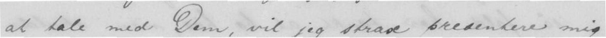at tale med Dem, vil jeg strax presentere mig
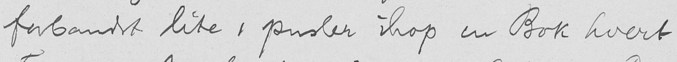forbandet lite, pusler ihop en Bok hvert
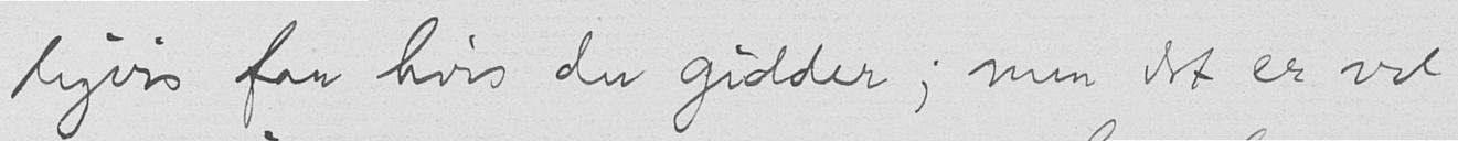ligvis faa hvis du gidder; men det er vel
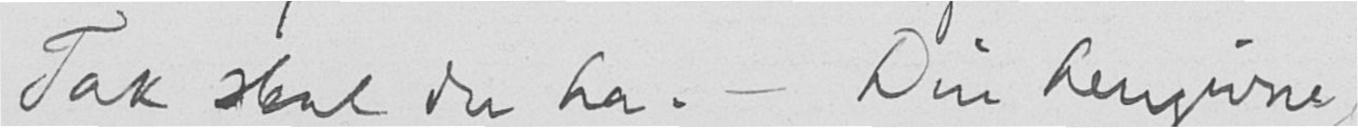Tak skal du ha. - Din hengivne
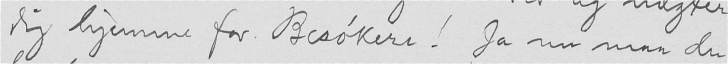dig hjemme for Besøkere! Ja nu maa du
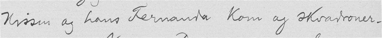Nissen og hans Fernanda kom og skvadroner-
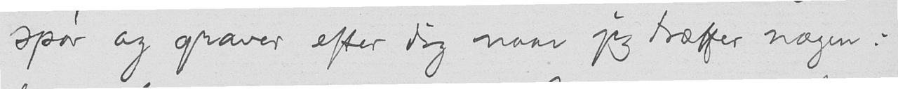spør og graver efter dig naar jeg træffer nogen:
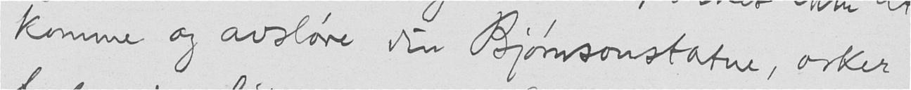komme og avsløre din Bjørnsonstatue, orker
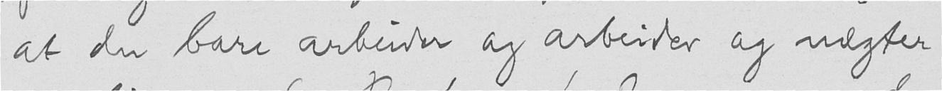at du bare arbeider og arbeider og nægter
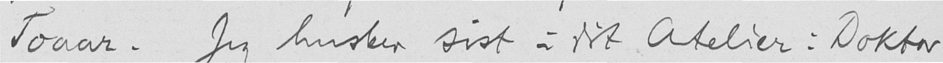Toaar. Jeg husker sist i dit Atelier: Doktor
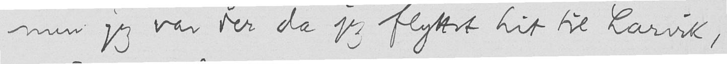men jeg var der da jeg flyttet hit til Larvik,
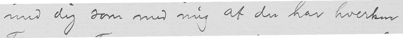med dig som med mig at du har hverken
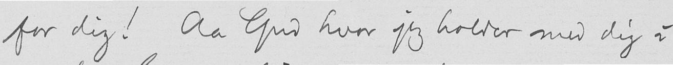for dig! Aa Gud hvor jeg holder med dig i
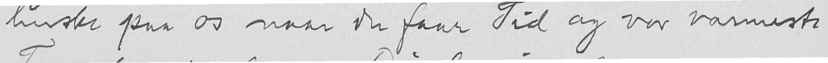huske paa os naar du faar Tid og vor varmeste
